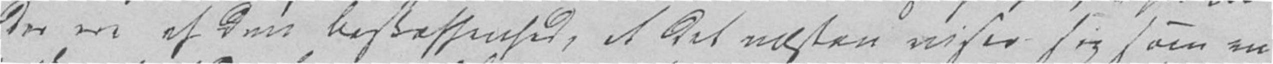der ere af den Beskaffenhed, at det næsten viser sig som en
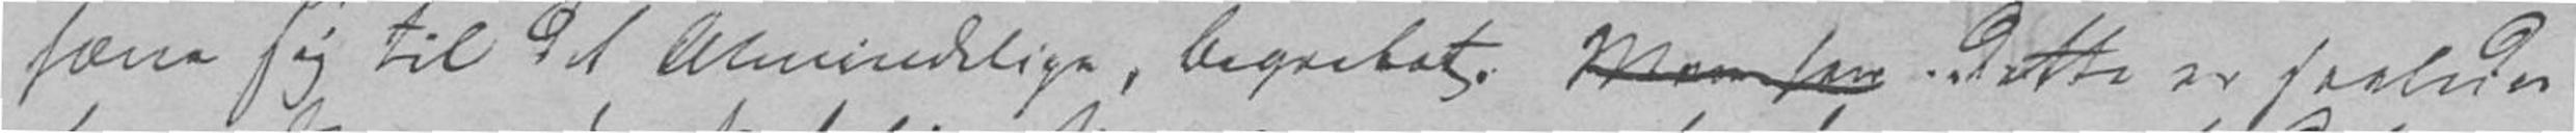hæve sig til det Almindelige, Begrebet. Men han Dette er saaledes
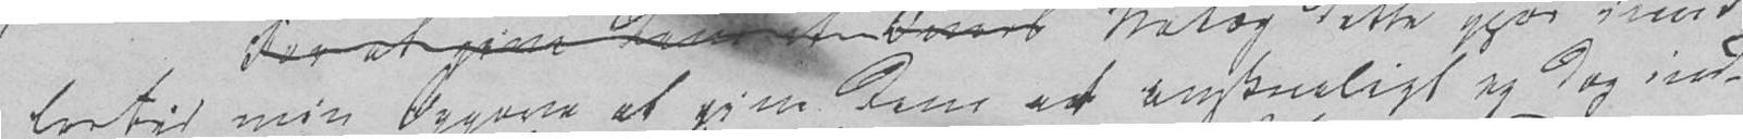lertid min Opgave at give Dem et anskueligt og dog ind-
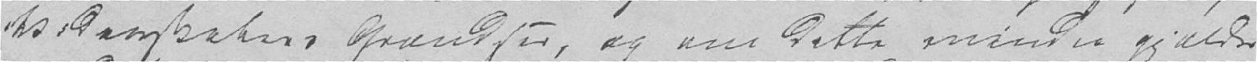Videnskabers Grændser, og om dette mindre gjælder
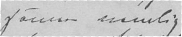savner nemlig
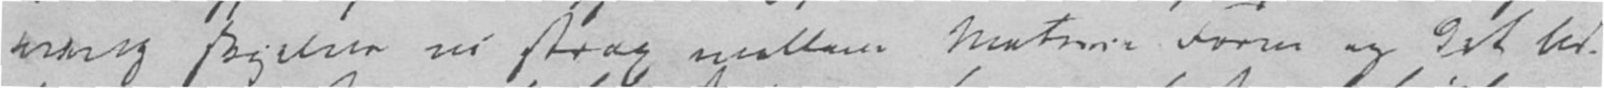ning skjelner vi strax mellem Materie Form og det Ud-
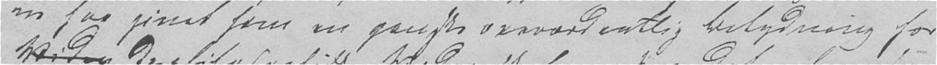ne har givet ham en ganske overordentlig Betydning for
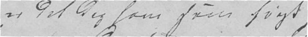er det dog han som først
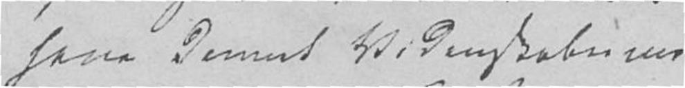have dannet Videnskabernes
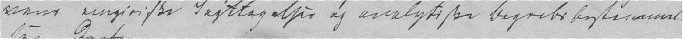nem empiriske Iagttagelser og analytiske Begrebsbestemmel-
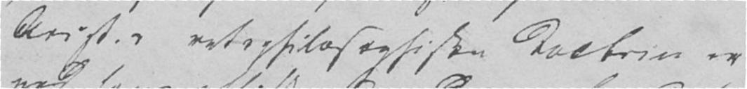Arist. retsphilosophiske Doctrin er
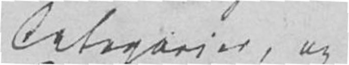Categorier, og
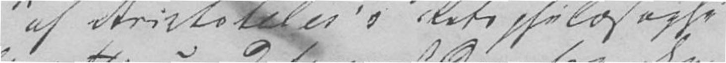af Aristoteles's Retsphilosophi
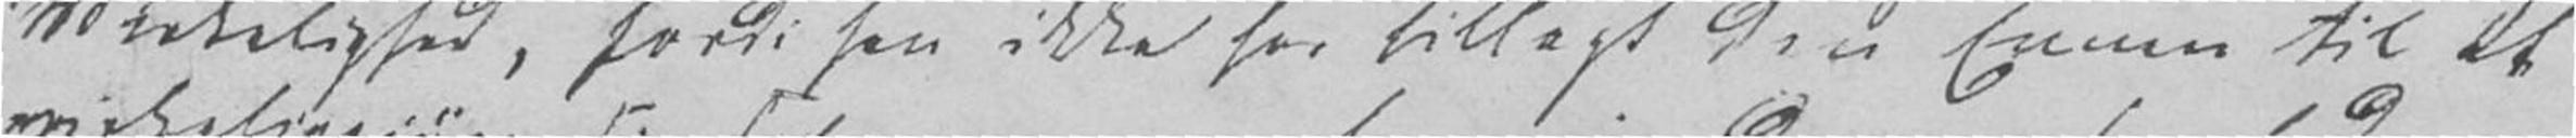Virkelighed, fordi han ikke har tillagt den Evnen til at
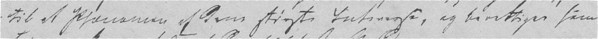til et Phænomen af den største Interesse, og berettiger ham
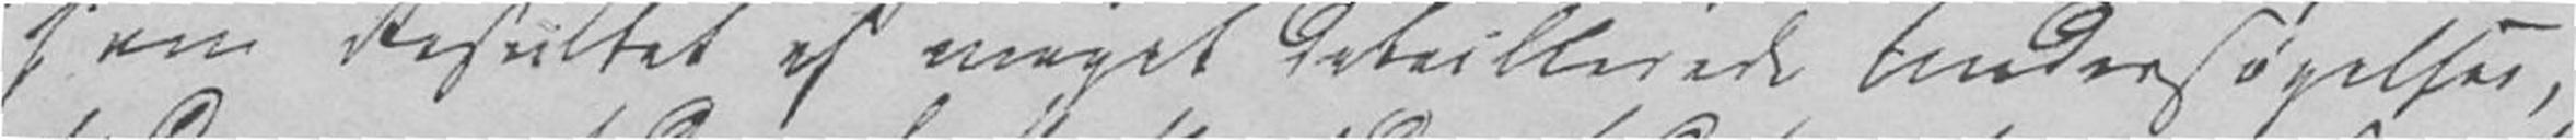som Resultat af meget detaillerede Undersøgelser,
